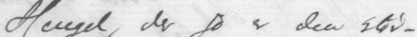Heugel, der jo er den -
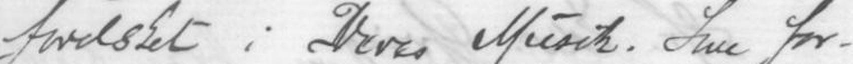forelsket i Deres musik. Som for-
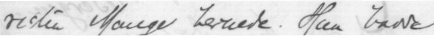resten Mange hernede. Han havde
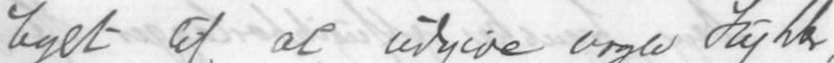lyst til at udgive nogle Stykker
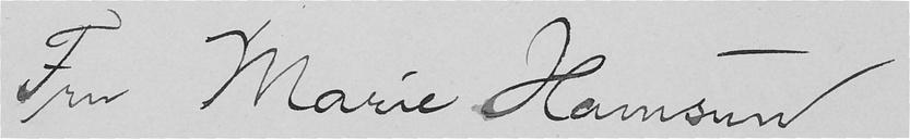Fru Marie Hamsun
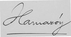Hamarøy
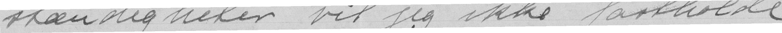stændigheter vil jeg ikke fastholde
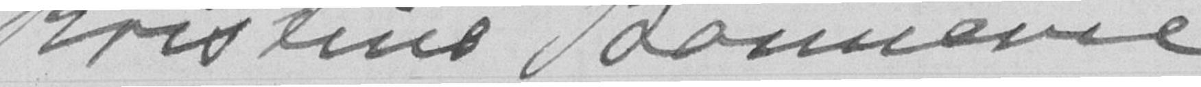Kristine Bonnevie
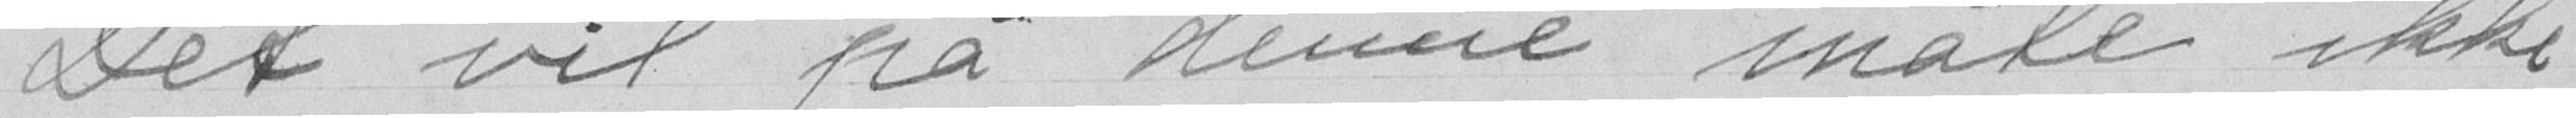Det vil på denne måte ikke
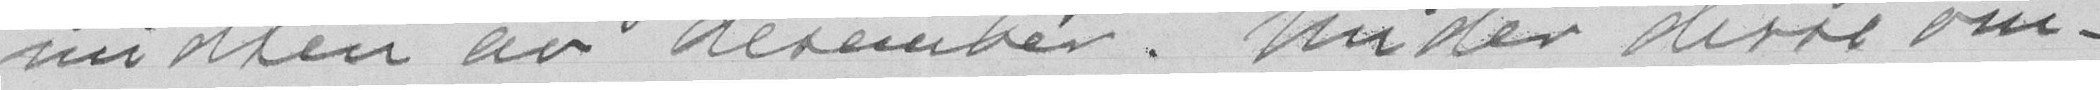midten av desember. Under disse om-
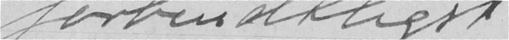forbindtligst
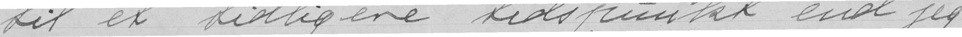til et tidligere tidspunkt end jeg
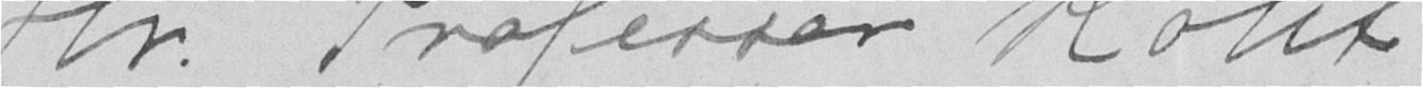Hr. Professor Koht
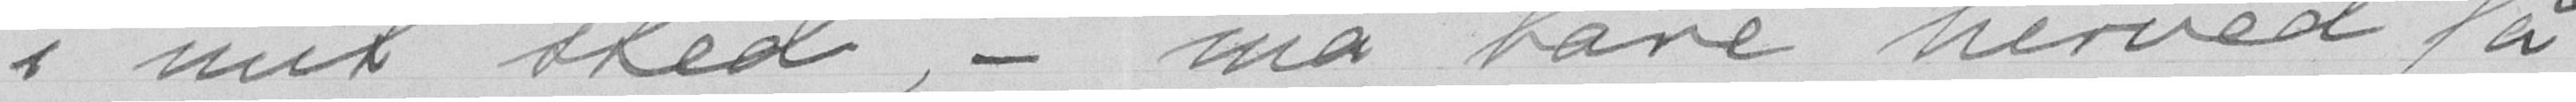i mit sted, - må bare herved få
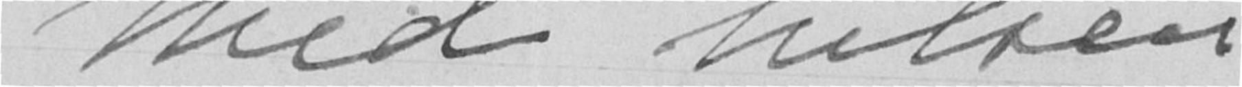Med hilsen
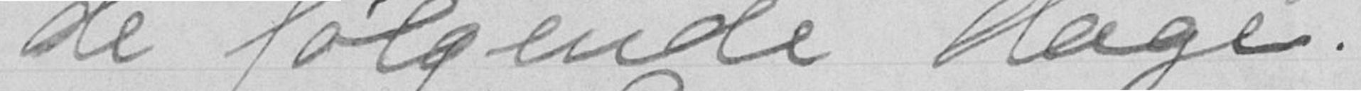de følgende dage.
